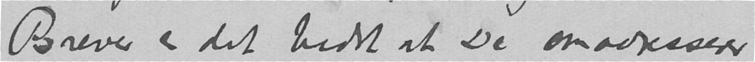Brever er det bedst at De omadresserer
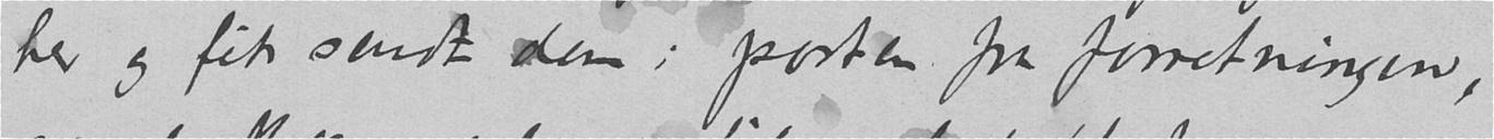her og fik sendt dem i posten fra forretningen,
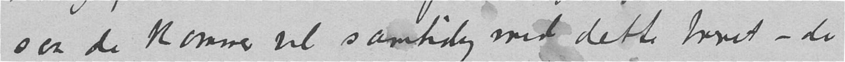saa de kommer vel samtidig med dette brevet – de
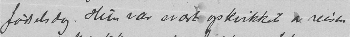fødselsdag. Hun var svært opkvikket av reisen
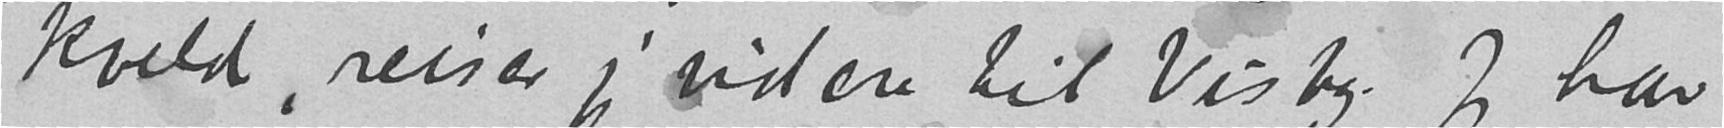kveld, reiser jeg videre til Visby. Jeg har
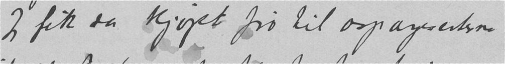Jeg fik da kjøpt frø til aspargeserterne
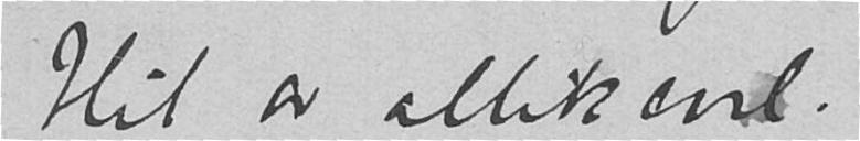blit av allikevel.
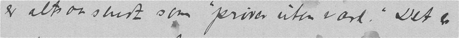er altsaa sendt som "prøver uten værd." Det er
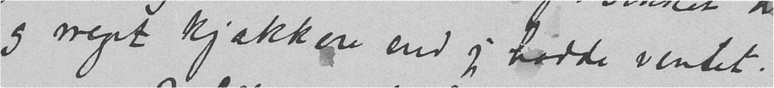og meget kjækkere end jeg nadde ventet.
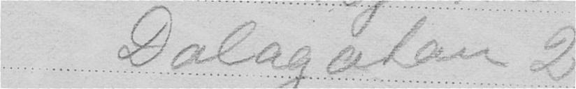Dalagatan 22
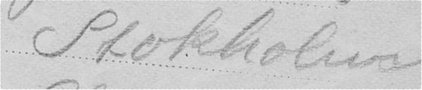Stokholm
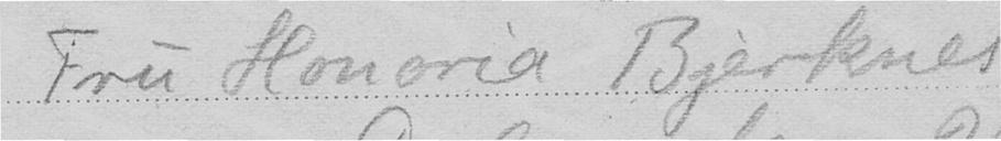Fru Honoria Bjerknes
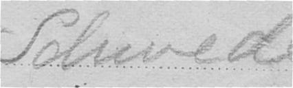Schweden
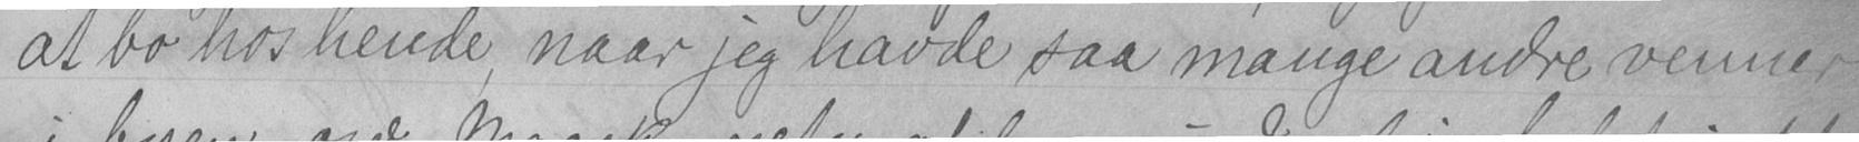at bo hos hende, naar jeg havde saa mange andre venner
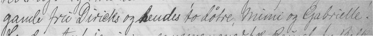gamle fru Diricks og hendes to døtre, Mimi og Gabrielle.
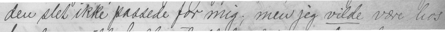den slet ikke passede for mig; men jeg vilde være hos
